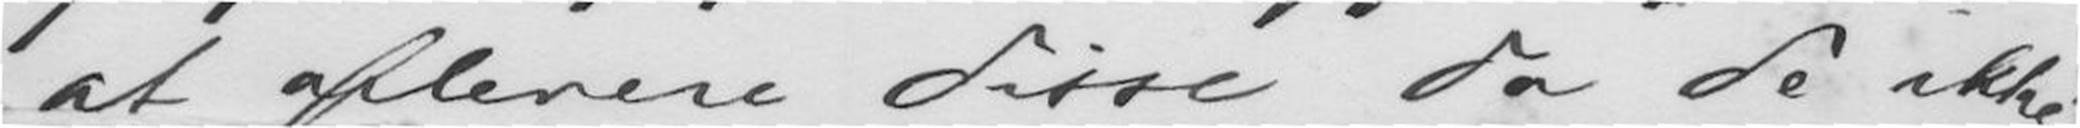at aflevere disse da de ikke
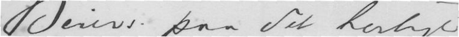Beiers paa dit herlige
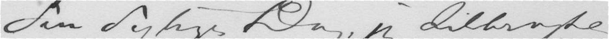den deilige Dag, jeg tilbragte
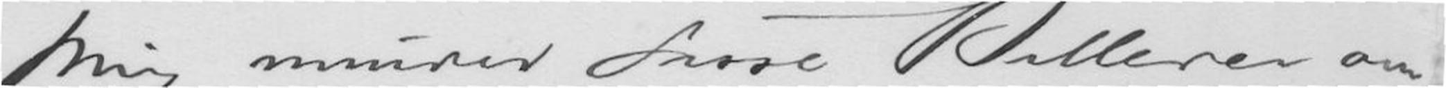Mig minder disse Billeder om
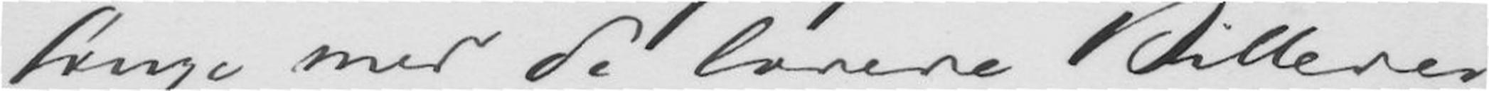længe med de lovede Billeder
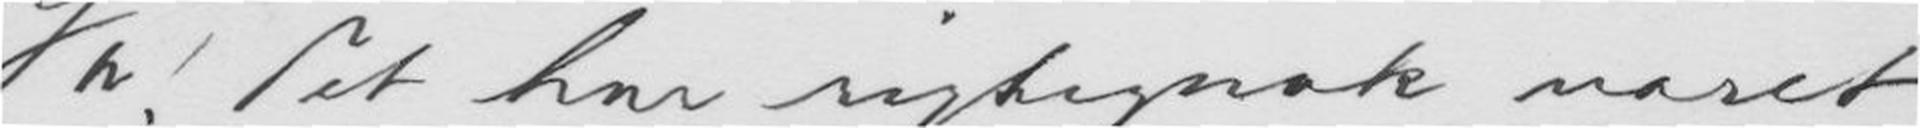Ja! Det har rigtignok varet
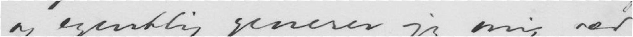og egentlig generer jeg mig ved
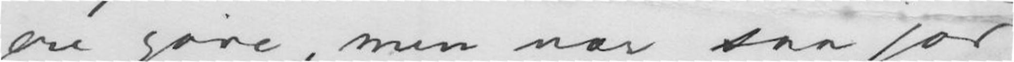ere gave, men vær saa god
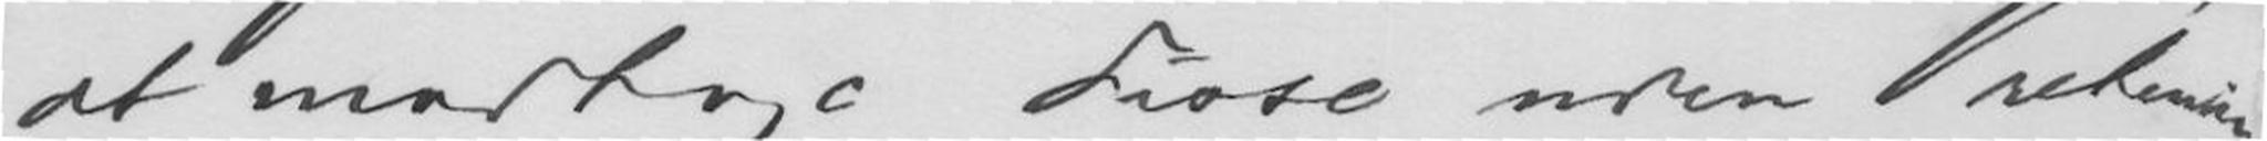at modtage disse uden Pretension
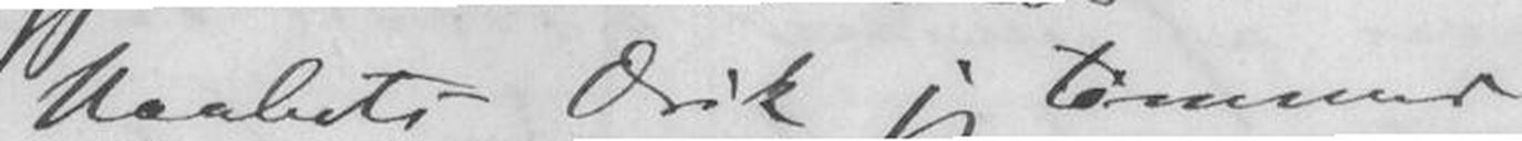Haabets Drik jeg tømmer
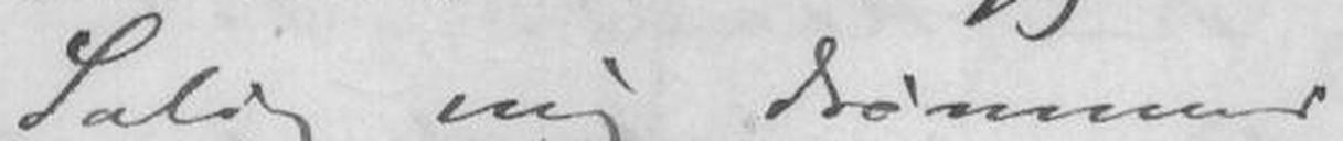Salig mig drømmer
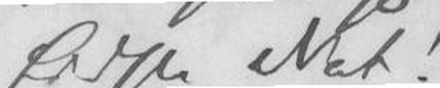Sidste Nat!
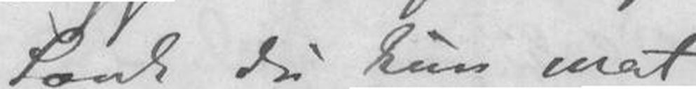Sank du kun mat
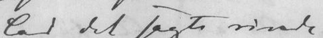Lad det sagte rinde
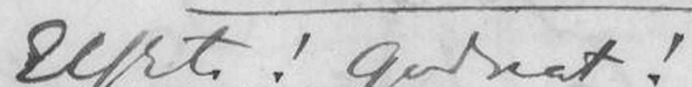Elskte! Godnat!
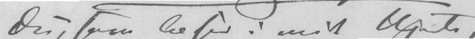Du, som læser i mit Hjerte,
